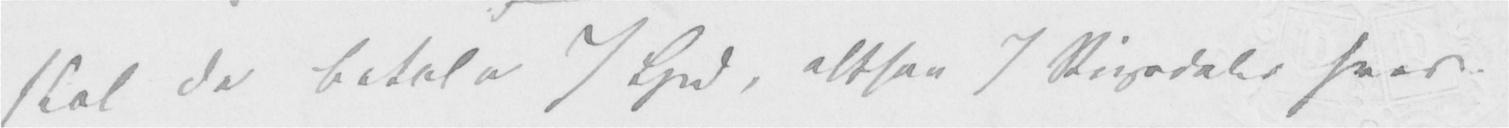skal de betale 7 Spd, altsaa 7 Rigsdaler hver.
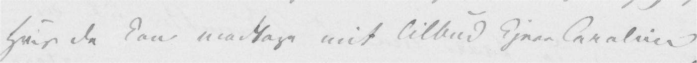Hvis De kan modtage mit Tilbud kjere Caroline
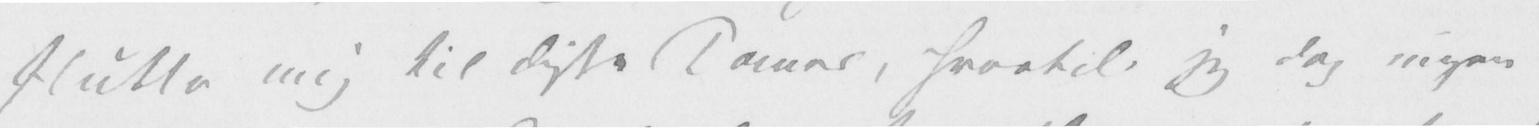slutte mig til disse Damer, hvortil jeg dog ingen
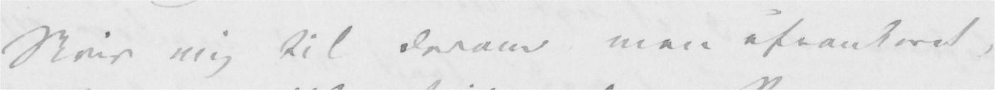Skriv mig til derom men ufrankeret,
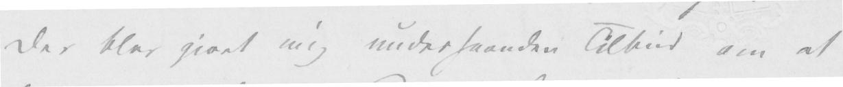Der blev gjort mig underhaanden Tilbud om at
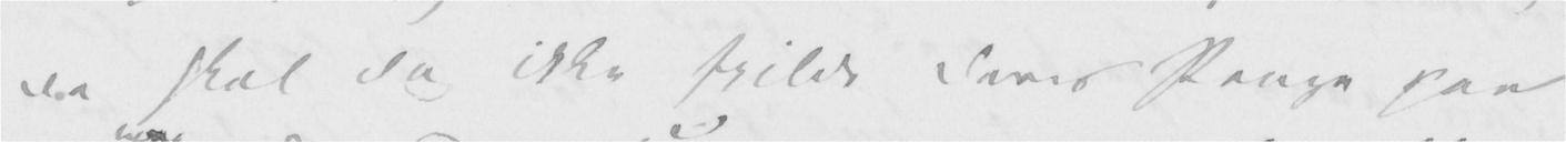De skal dog ikke spilde Deres Penge paa
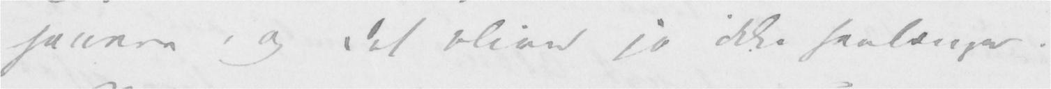senere, og det bliver jo ikke saalænge.
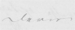Deres
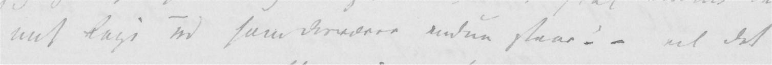mit Logi ud som desværre endnu staar – – vil det
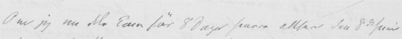Om jeg nu ikke kom før 8 Dage senere altsaa den 8de Juni
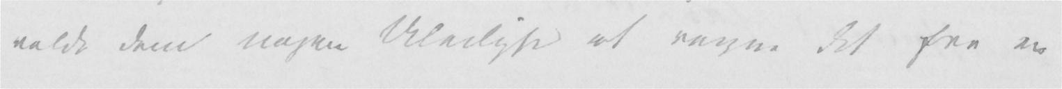volde Dem nogen Uleilighed at regne det fra en
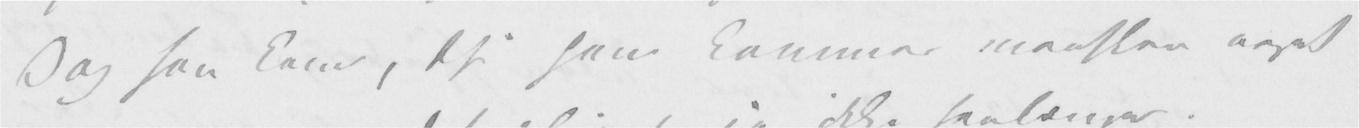Dag han kom, thi han kommer maaskee noget
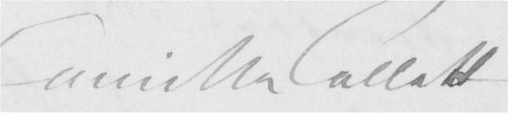Camilla Collett
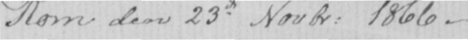Rom den 23de Novbr: 1866.-
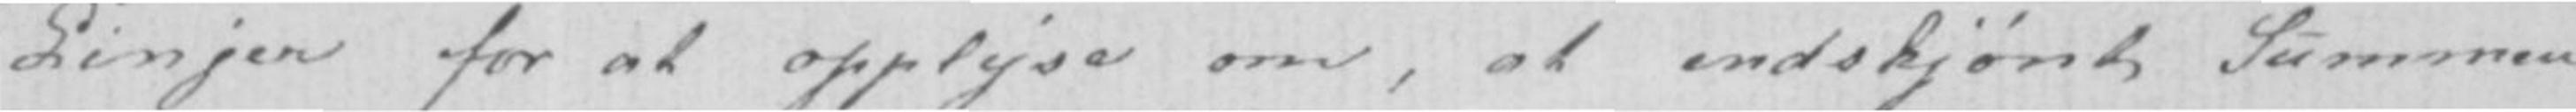Linjer for at opplyse om, at endskjønt Summen
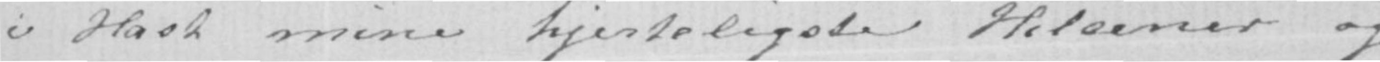i Hast mine hjerteligste Hilsener og
